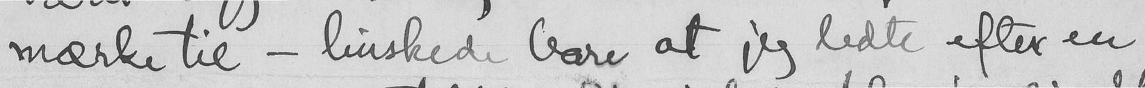mærke til - huskede bare at jeg ledte efter en
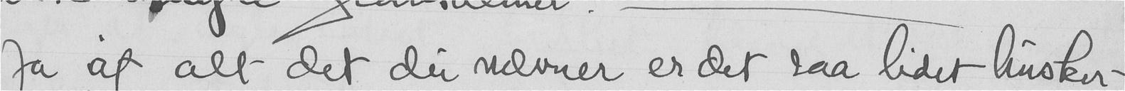Ja af alt det du nævner er der saa lidet husker -
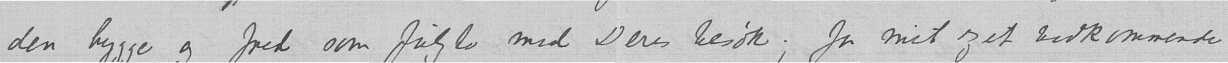den hygge og fred som fulgte med Deres besøk; for mit eget vedkommende
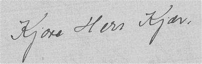Kjære Herr Kjær,
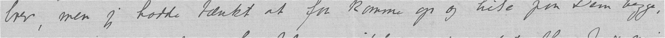brev, men jeg hadde tænkt at faa komme op og hilse paa Dem begge,
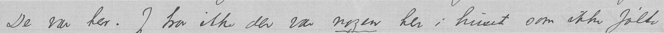De var her. Jeg tror ikke der var nogen her i huset som ikke følte
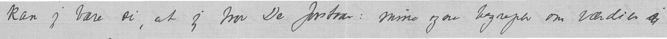kan jeg bare si, at jeg tror De forstaar: mine egne begreper om værdier i
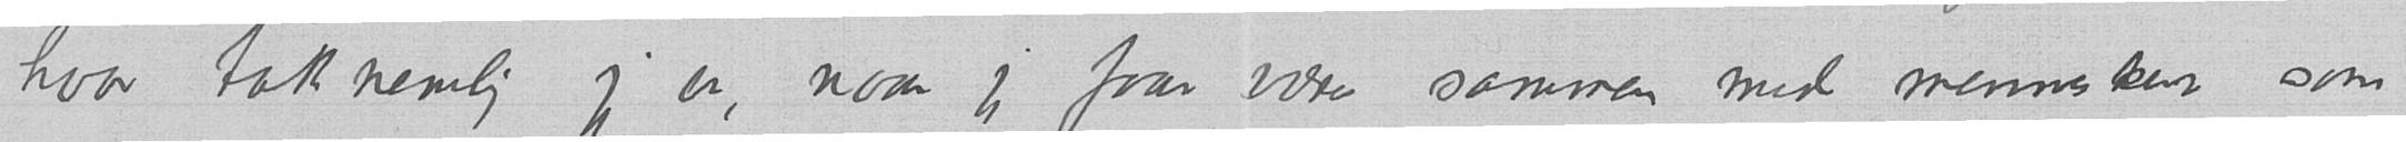hvor taknemlig jeg er, naar jeg faar være sammen med mennesker som
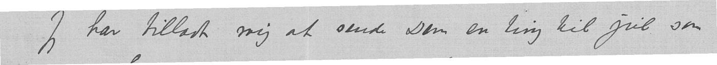Jeg har tilladt mig at sende Dem en ting til jul, som
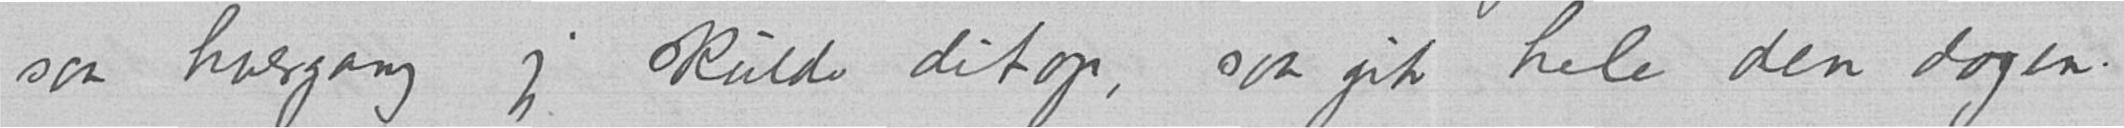saa hvergang jeg skulde ditop, saa gik hele den dagen.
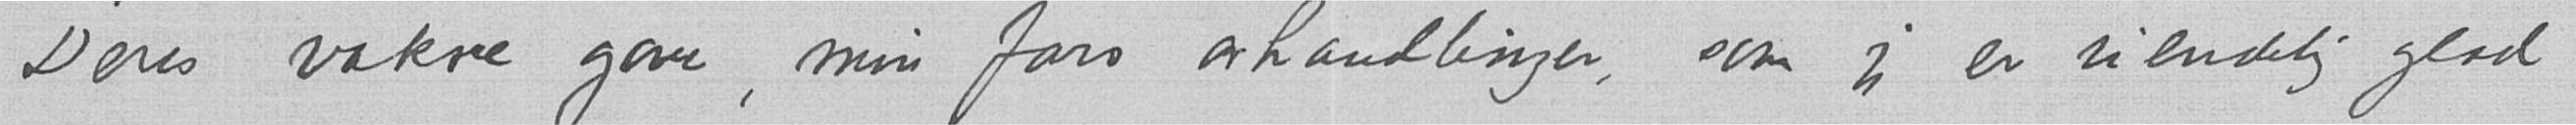Deres vakre gave, min fars avhandlinger, som jeg er uendelig glad
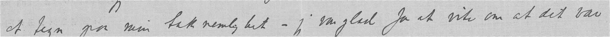et tegn paa min taknemlighet – jeg var glad for at vite om at det var
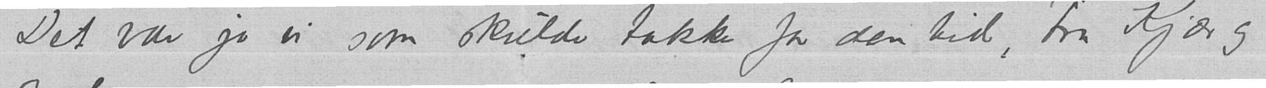Det var jo vi som skulde takke for den tid, Fru Kjær og
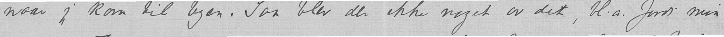naar jeg kom til byen. Saa blev der ikke noget av det, bl.a. fordi min
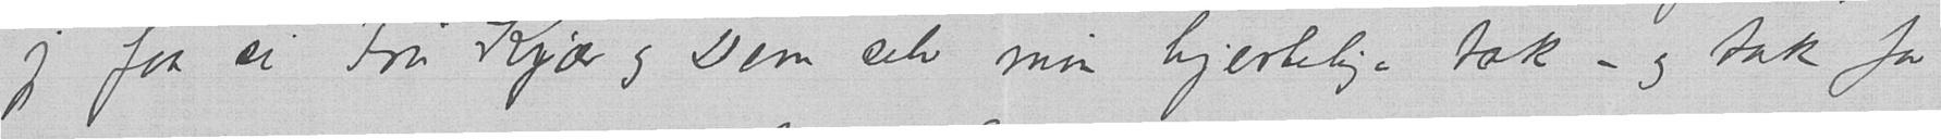jeg faa si Fru Kjær og Dem selv min hjertelige tak – og tak for
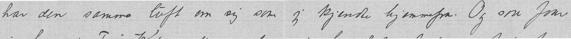har den samme luft om sig som jeg kjendte hjemmefra. Og saa faar
